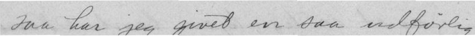- saa har jeg givet en saa udførlig
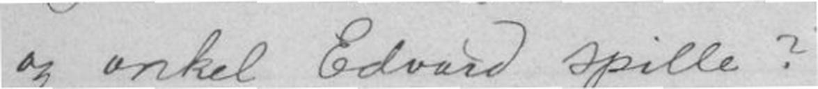og onkel Edvard spille?
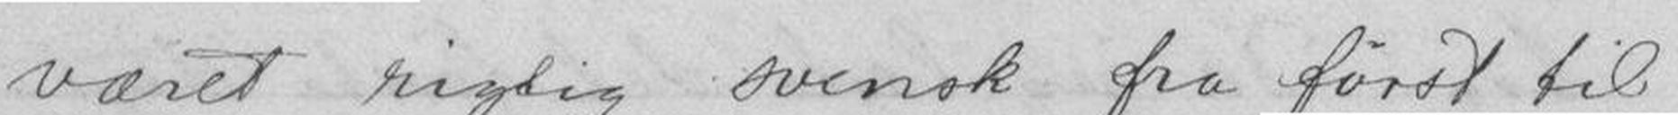været rigtig svensk fra først til
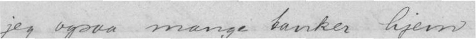jeg ogsaa mange tanker hjem -
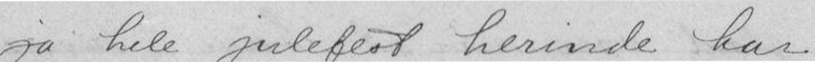- ja hele julefest herinde har
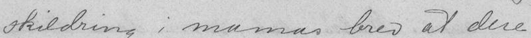skildring i mamas brev at dere
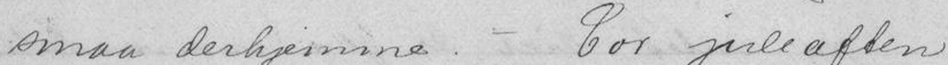smaa derhjemme. - Vor juleaften
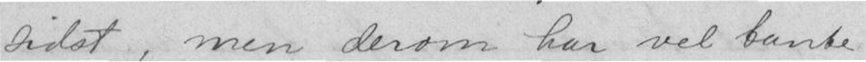sidst, men derom har vel tante
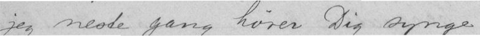jeg neste gang hører Dig synge
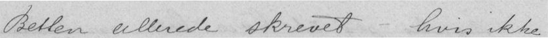Betten allerede skrevet - hvis ikke
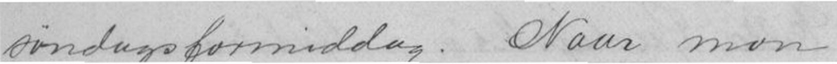søndagsformiddag. Naar mon
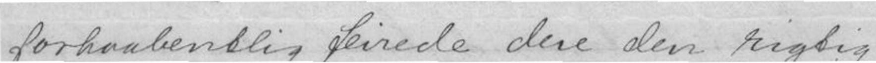forhaabentlig feirede dere den rigtig
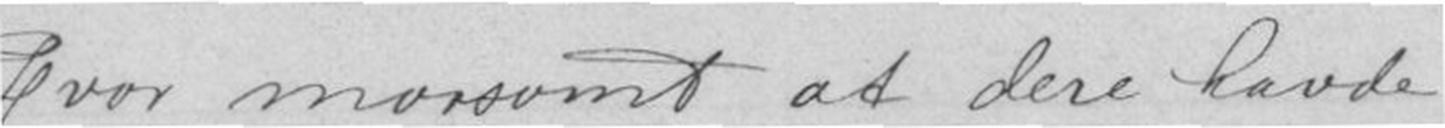-Hvor morsomt at dere havde
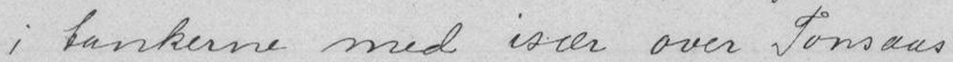i tankerne med især over Tonsaas
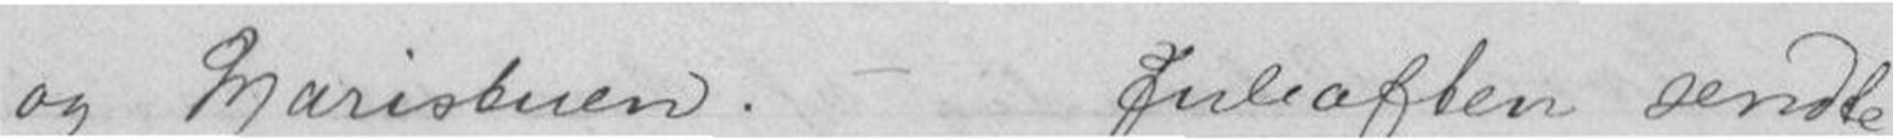og Maristuen. - Juleaften sendte
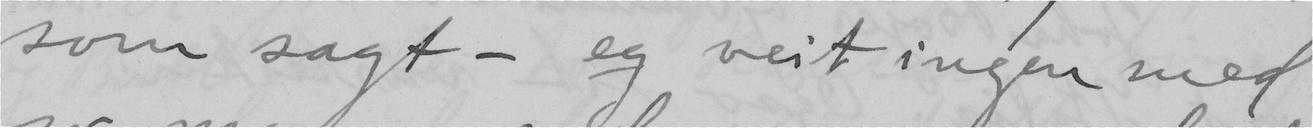som sagt – eg veit ingen med
